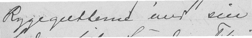Roggegutterne med sin
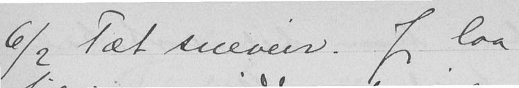6/2 Tæt sneveir. Jeg laa
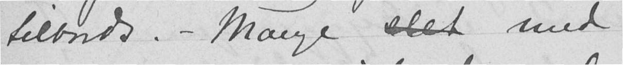tilbords. - Mange slet med
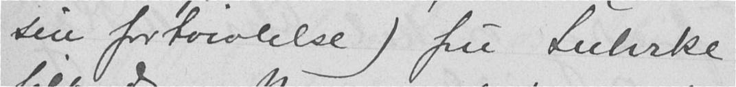sin fortvivlelse) fru Suhrke
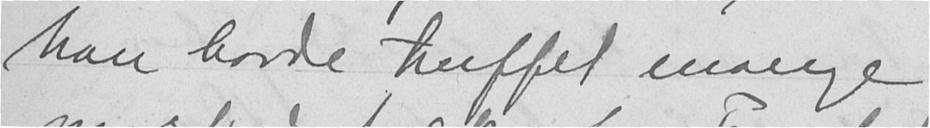han havde truffet mange
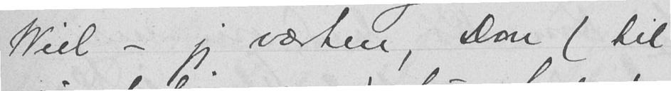Wiel - jeg værten, Don (til
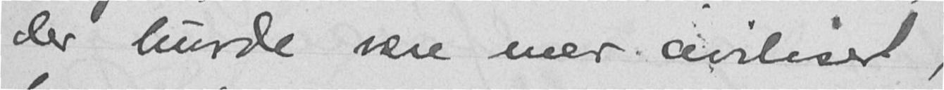der burde være mer civilisert,
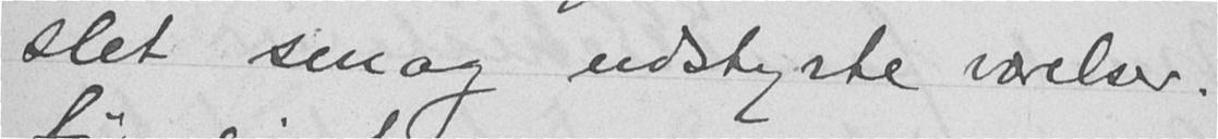slet smag udstyrte værelser.
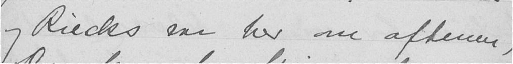og Riecks var her om aftenen,
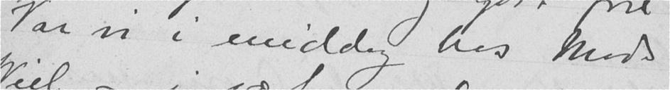Var vi i middag hos Mads
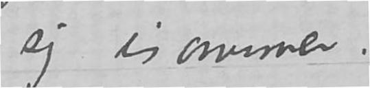sig isommer.
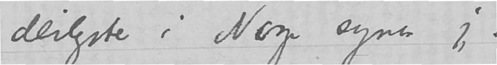deiligste i Norge synes jeg.
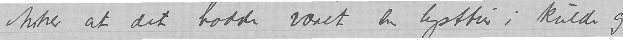Anker at det hadde været en lysttur i kulde og
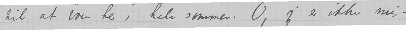til at være her i hele sommer.  Og jeg er ikke mis
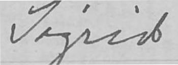Sigrid
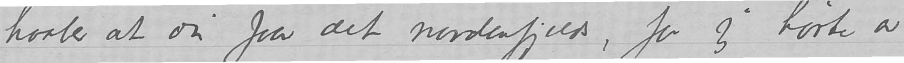hørte at du faar det nordenfjelds, for jeg hørte av
